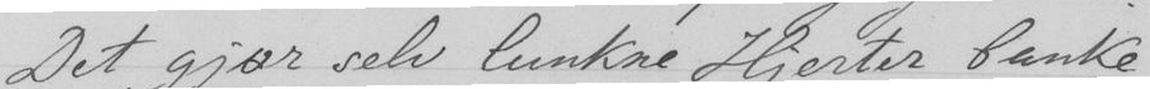Det gjør selv lunkne Hjerter banke
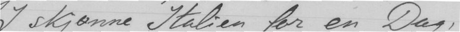I skjønne Italien for en Dag,
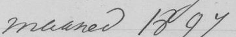maaned 1897
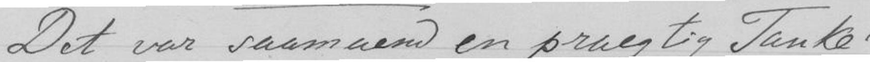Det var saamaend en praegktig Tanke!
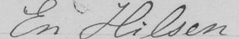En hilsen
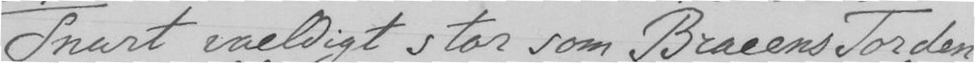Snart vaeldigt stor som Braens Torden
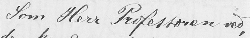Som Herr Professoren ved,
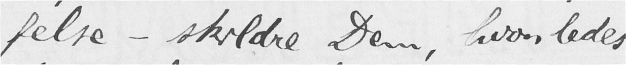felse - skildre Dem, hvorledes
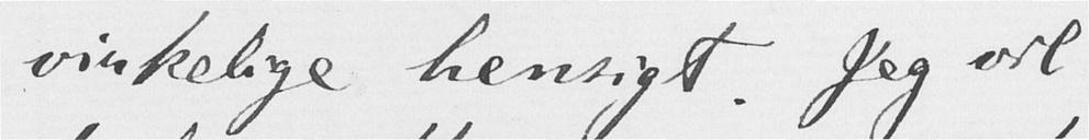virkelige hensigt. Jeg vil
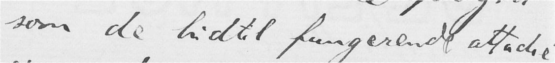som de hidtil fungerende attaché-
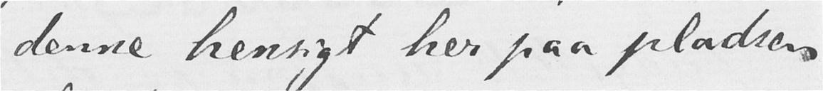denne hensigt her paa pladsen
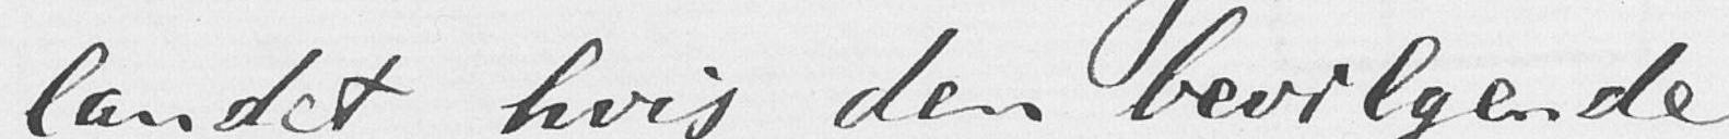landet, hvis den bevilgende
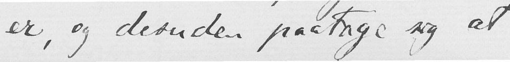er, og desuden paatage sig at
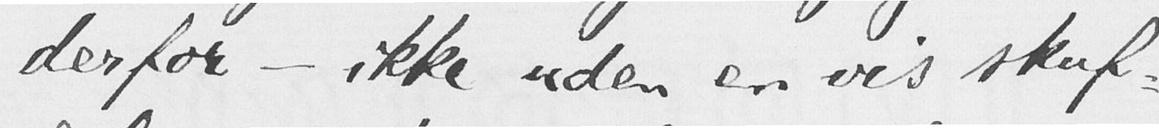derfor - ikke uden en vis skuf-
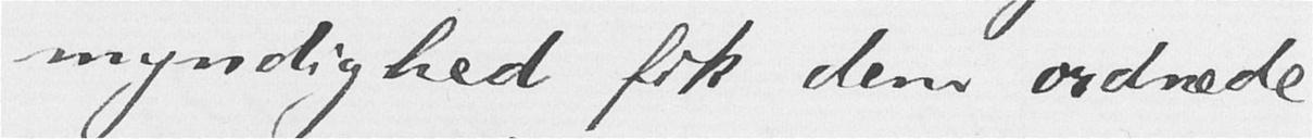myndighed fik dem ordnede
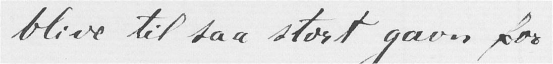blive til saa stort gavn for
